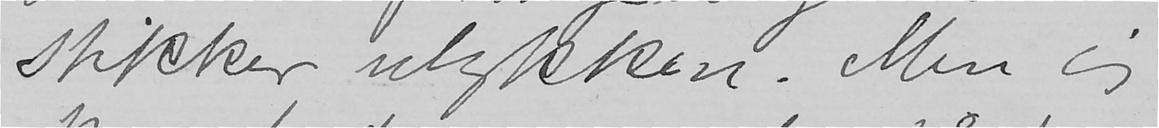stikker ulykken. Men jeg
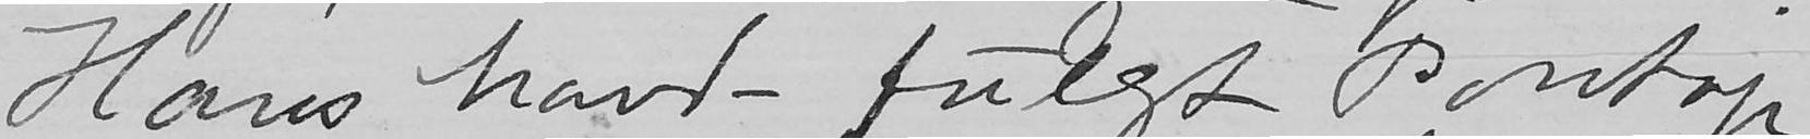Hans havde fulgt Pontop-
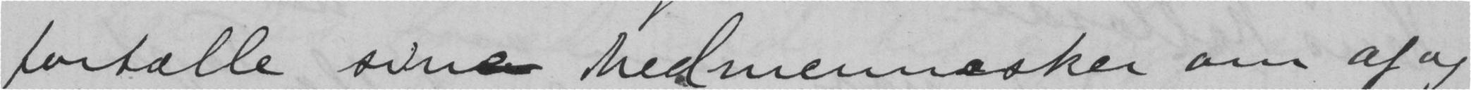fortælle sine Medmennasker om af og
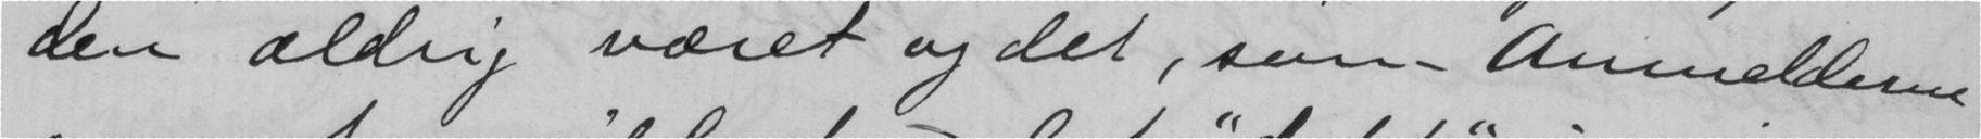den aldrig været og det, som anmelderne
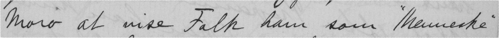moro at vise Folk ham som "Menneske"
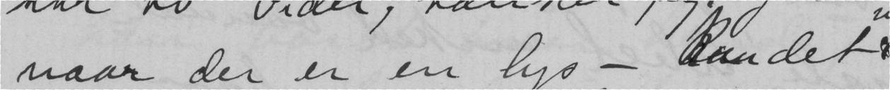naar der er en lys — kan det
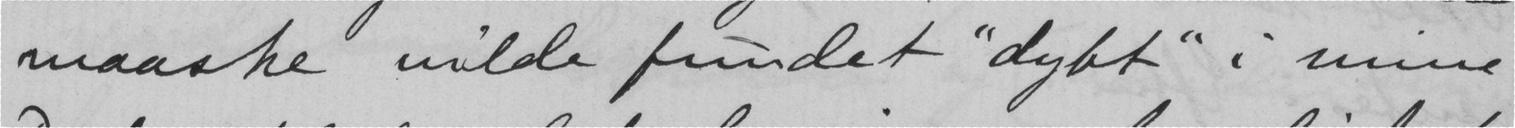maaske vilde fundet "dybt" i mine
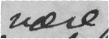være
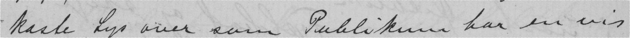kaste Lys over som Publikum har en vis
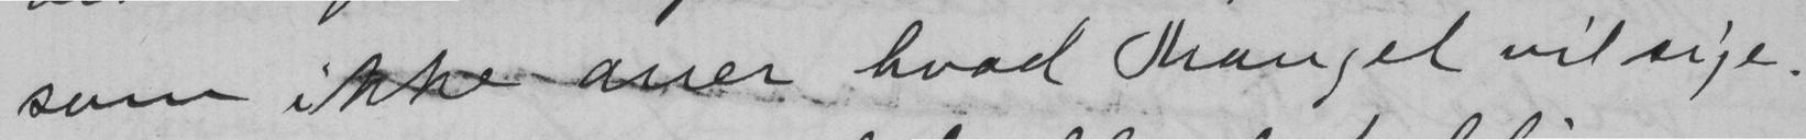som ikken aner hvad mangel vil sige!
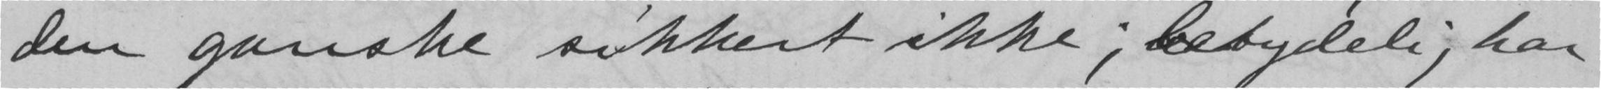den ganske sikkert ikke; bedydelig har
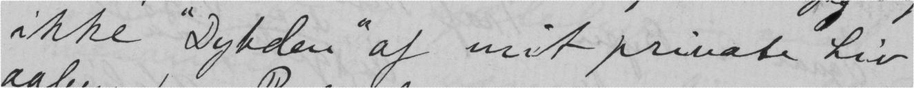ikke "Dybden" af mit private Liv
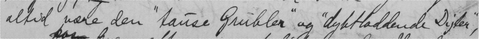altid være den "tause Grubler" og "dybtloddende Digter",
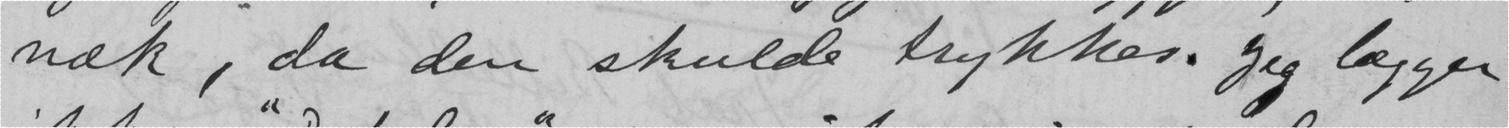væk, da den skulde trykkes. Jeg lægger
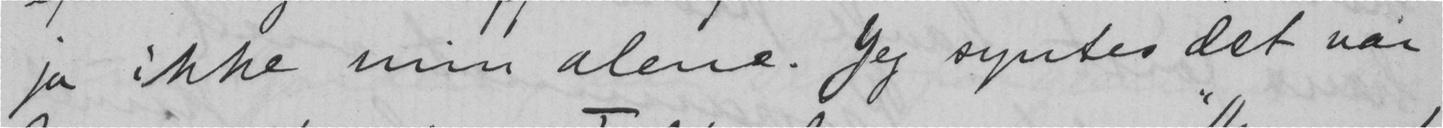jo ikke min alene. Jeg syntes det var
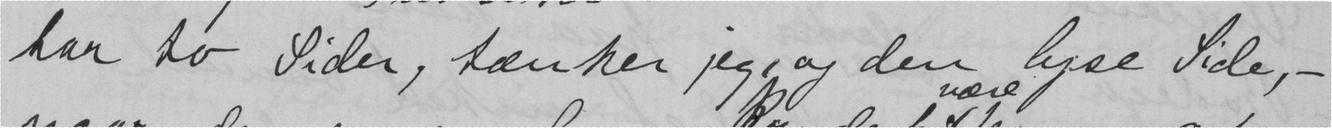har to Sider, tænker jeg, og den lyse Side,-
